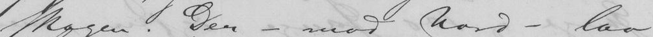skogen. Der - mod Nord - laa
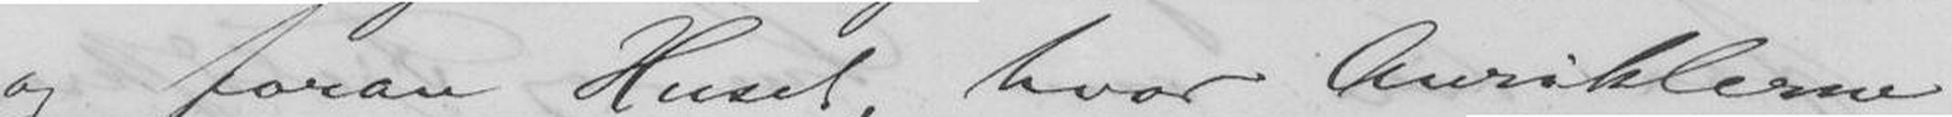og foran Huset, hvor Auriklerne
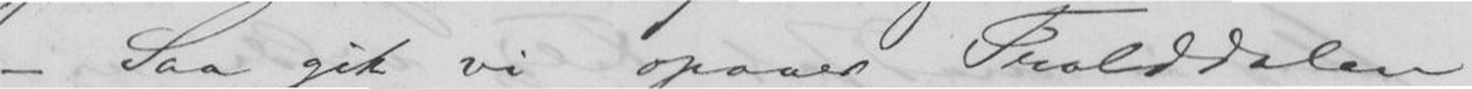- Saa gik vi opover Trolddalen
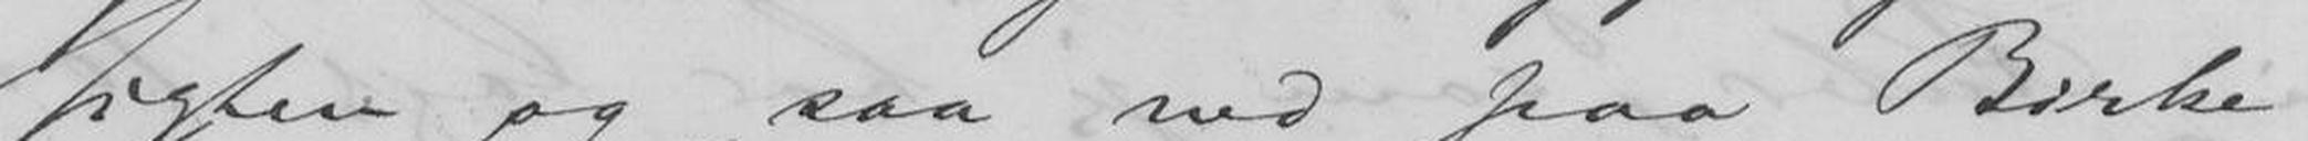sigten og saa ned paa Birke-
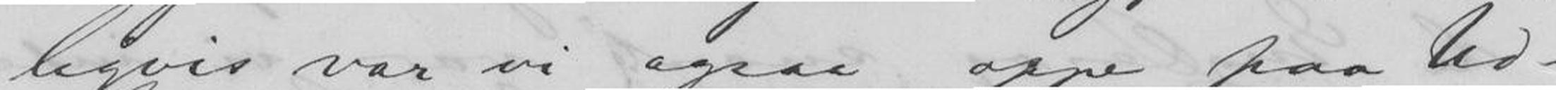ligvis var vi ogsaa oppe paa Ud-
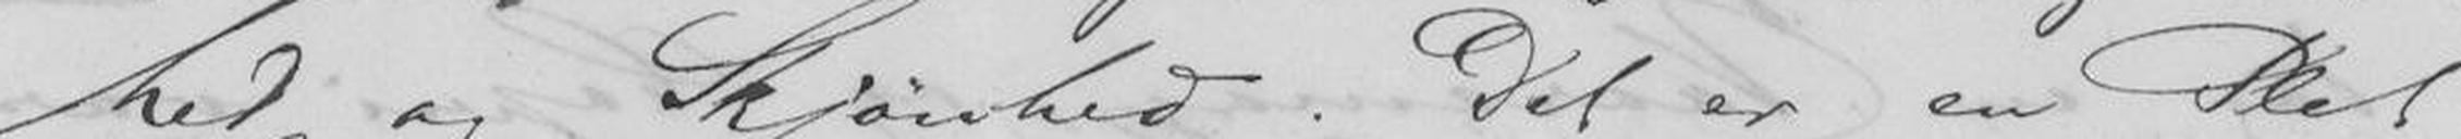hed og Skjønhed. Det er en Plet
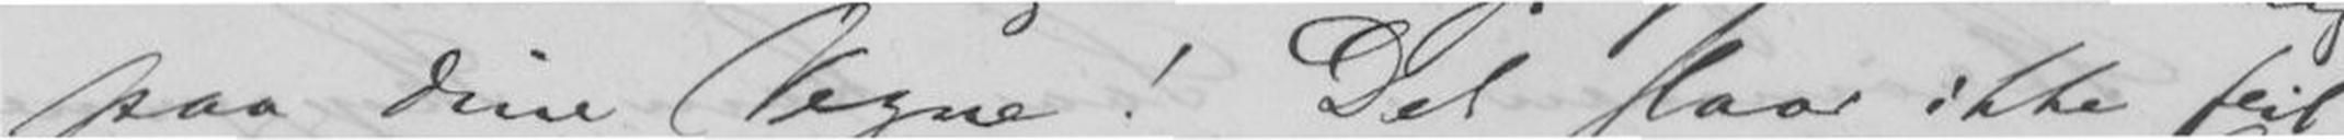paa Dine Vegne! Det slaar ikke feil,
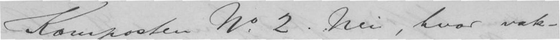Komposten N. 2. Nei, hvor vak-
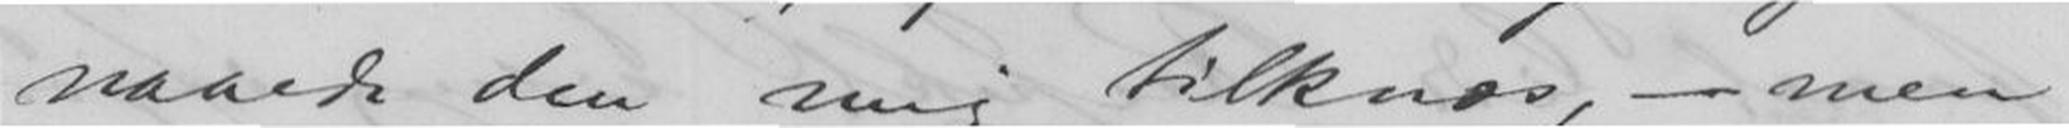naaede den mig tilknæs, - men
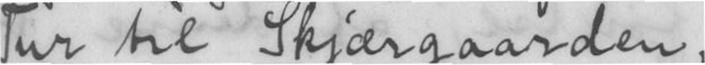Tur til Skjærgaarden,
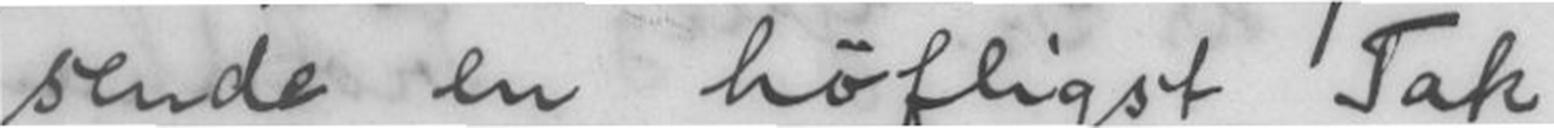sende en høfligst Tak
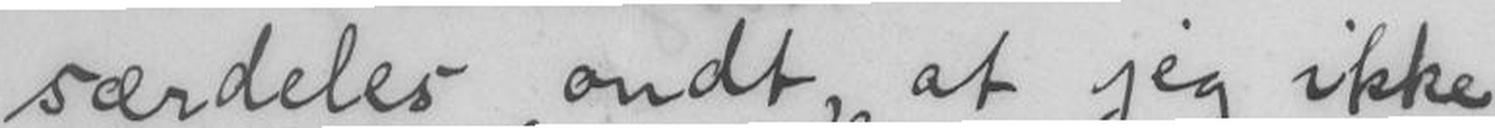særdeles ondt, at jeg ikke
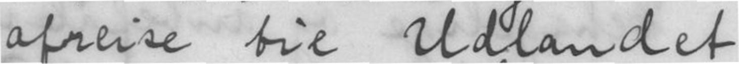afreise til Udlandet
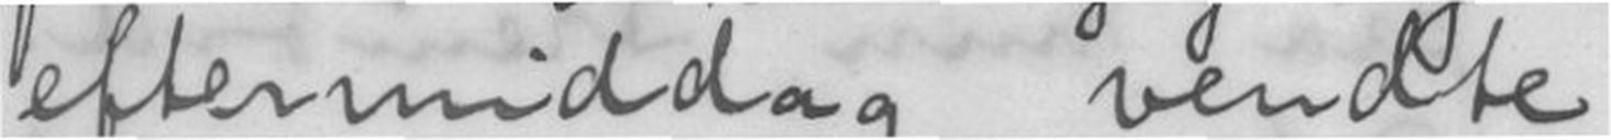eftermiddag vendte
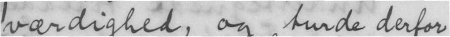værdighed, og turde derfor
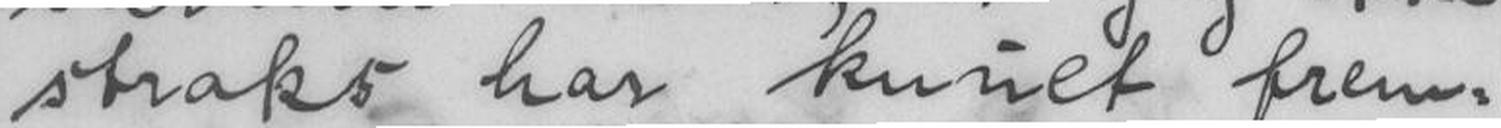straks har kunet frem-
